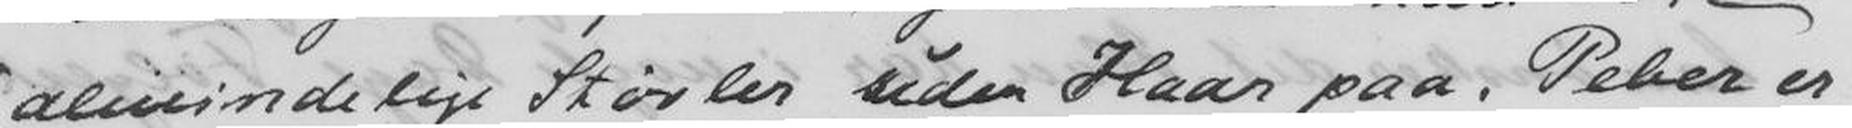almindelige Støvler uden Haar paa. Peber er
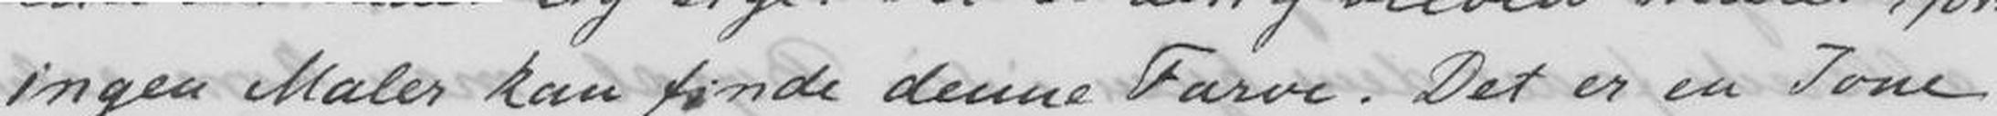ingen Maler kan finde denne Farve. Det er en Tone
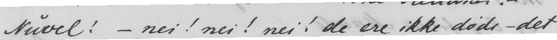Nuvel! - nei! nei! nei! de ere ikke døde - det
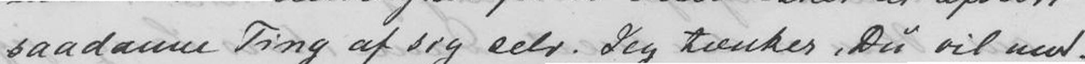afsløre saadanne Ting af sig selv. Jeg tænker, Du vil mod-
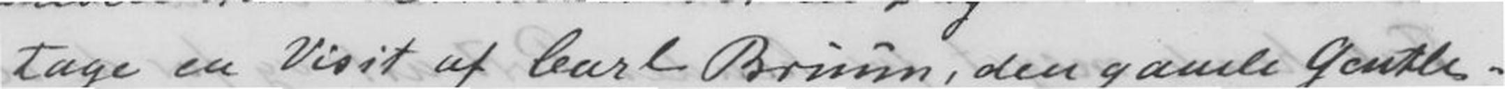Sommer at modtage en Visit af Carl Bruun, den
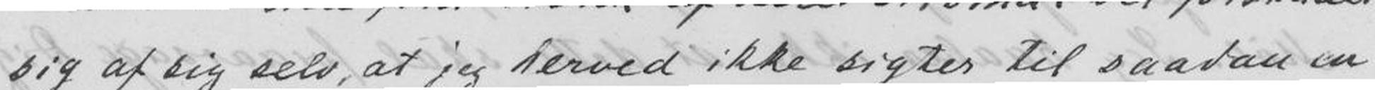sig af sig selv, at jeg derved ikke sigter til saadan en
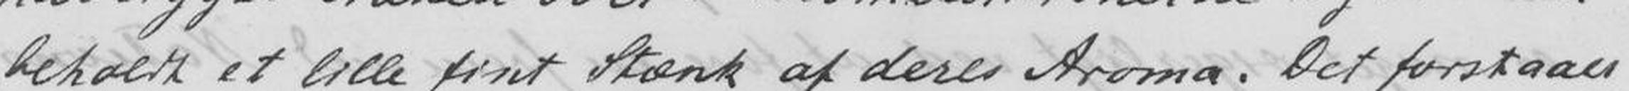beholdt et lille fint Stænk af deres Aroma. Det forstaaes
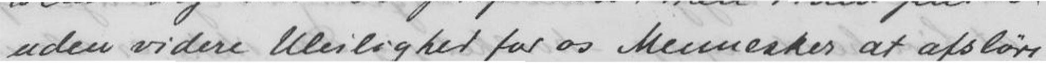uden videre Uleilighed for os Mennesker at
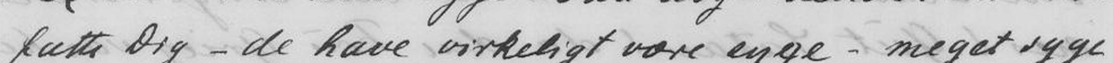fatte Dig - de have virkeligt være syge - meget syge
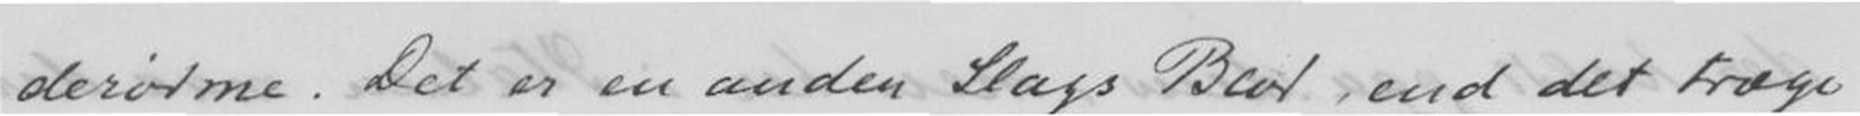derødme. Det er en anden Slags Blod end det træge
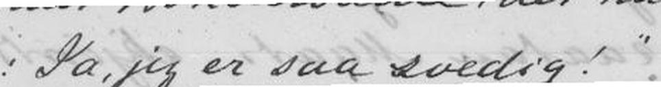Ja, jeg er saa svedig!
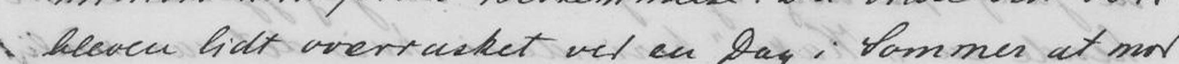bleven lidt overrasket ved en Dag i
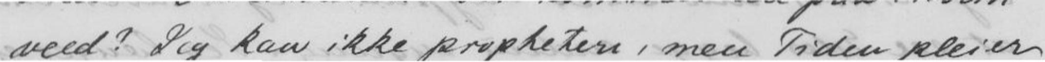Hvem veed? Jeg kan ikke prophetere, men Tiden pleier
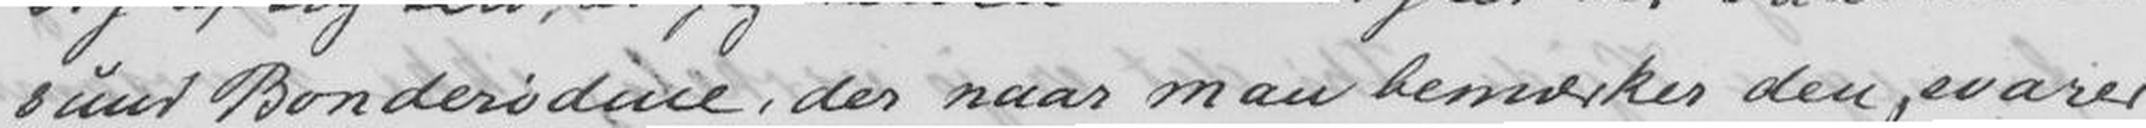sund Bonderødine, der naar man bemærker den, svarer
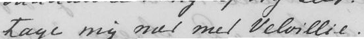tage mig med med Velvillie.
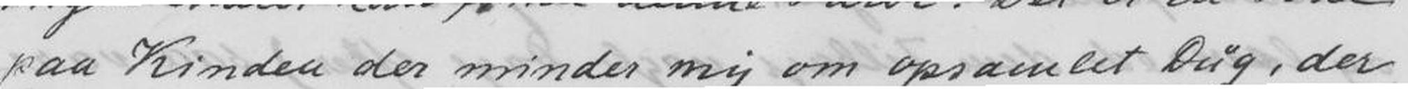paa Kvinden det mindes mig om opsamlet Dug, der
